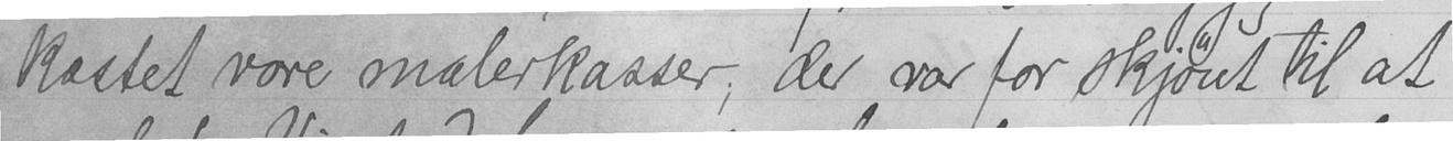kastet vore malerkasser; der var for skjønt til at
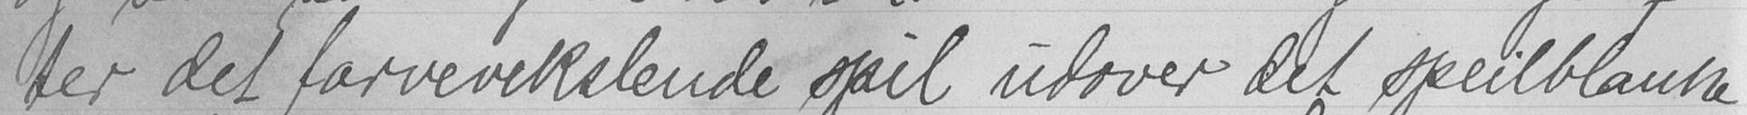ter det farvevekslende spil udover det speilblanke
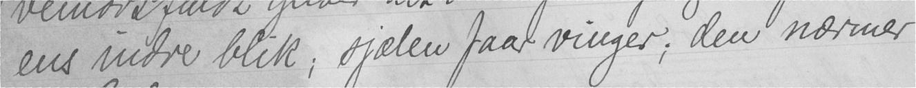ens indre blik; sjælen faar vinger; den nærmer
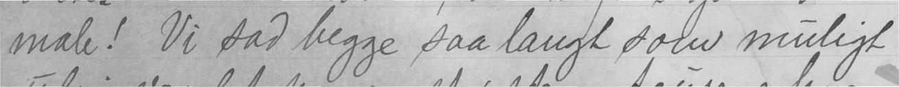male! Vi sad begge saa langt som muligt
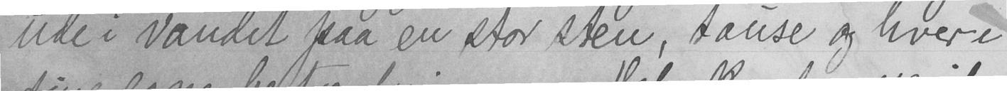ude i vandet paa en stor sten, tause og hver i
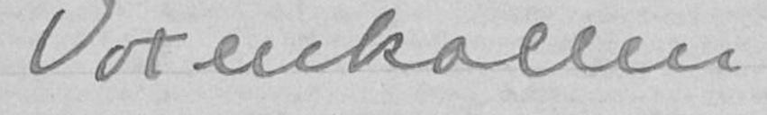Voxenkollen
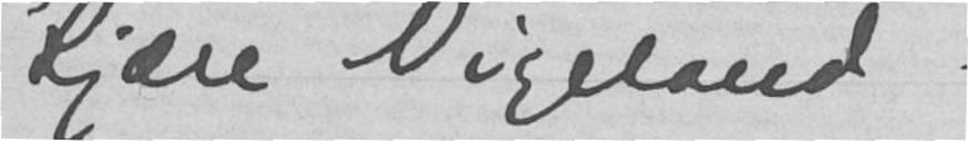Kjære Vigeland
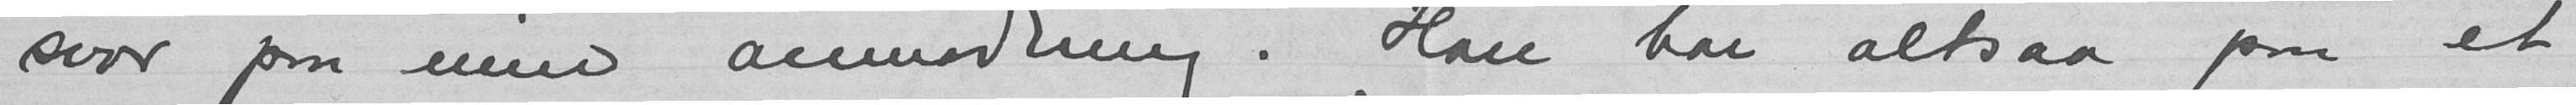svar paa min anmodning. Han har altsaa paa et
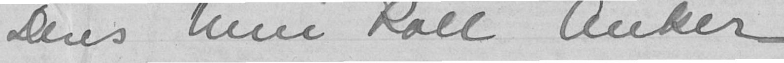Deres Nini Roll Anker
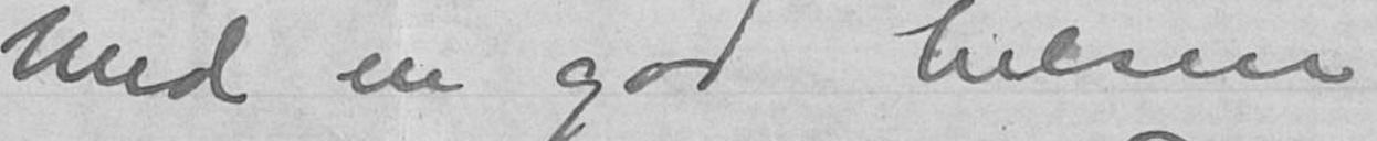Med en god hilsen
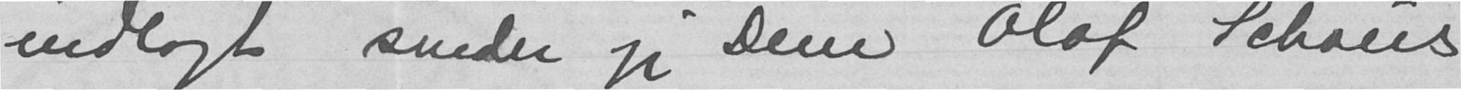indlagt sender jeg dem Olaf Schous
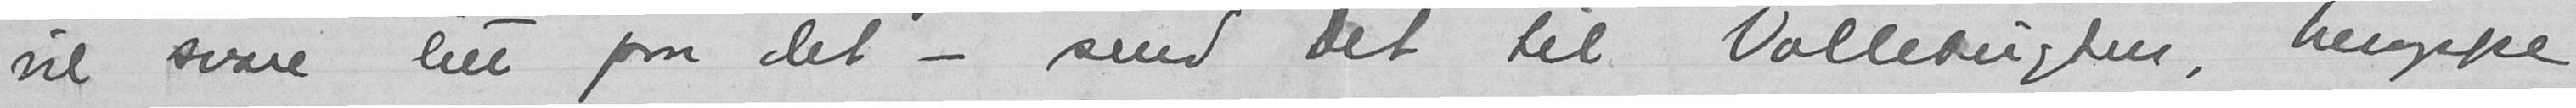vil svare litt paa det - send det til Vollebugten, heroppe
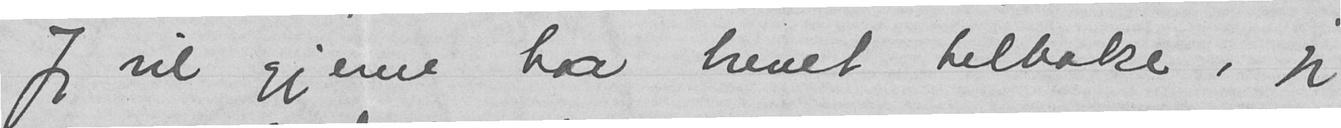Jeg vil gjerne ha brevet tilbake, jeg
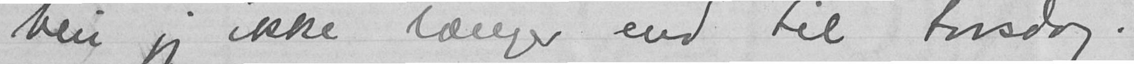blir jeg ikke længer end til torsdag.
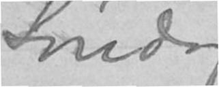Lørdag
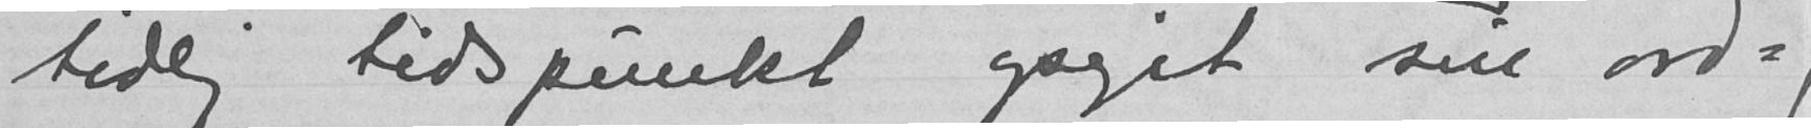tidlig tidspunkt opgit sin ord-
